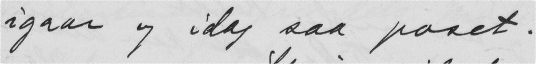igaar og idag saa poset.
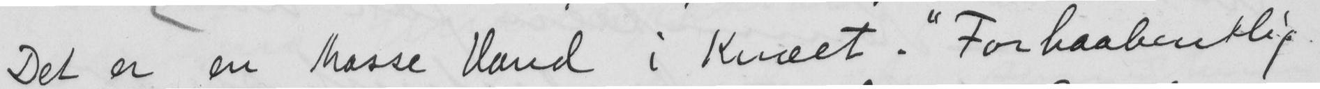Det er en Masse Vand i Knæet. "Forhaabentlig.
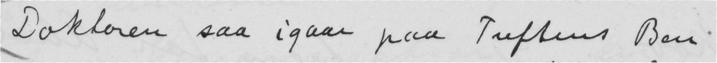Doktoren saa igaar paa Tuftens Ben
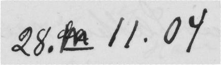28. 11.04
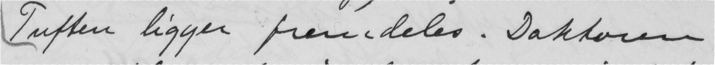Tuften ligger fremdeles. Doktoren
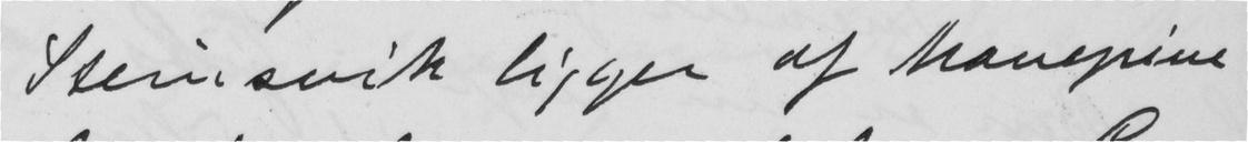Steinsvik ligger af Mavepine
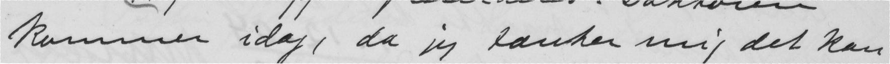kommer idag, da jeg tænker mig det kan
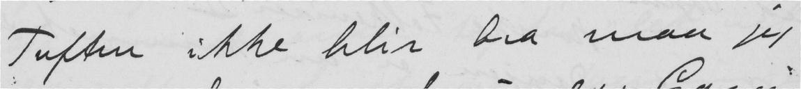Tuften ikke blir bra maa jeg
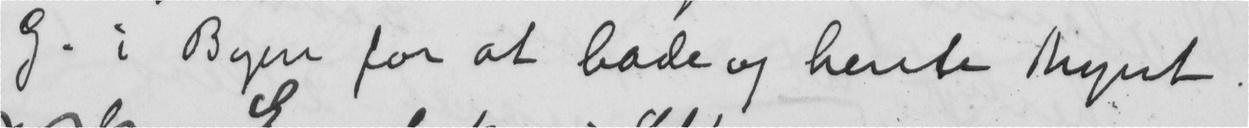G. i Byen for at bade og hente Mynt.
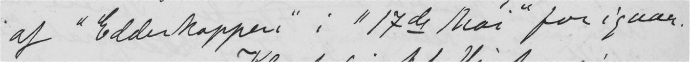af "EdderKoppen" i "17de Mai" for igaar.
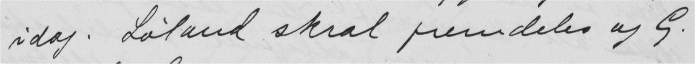idag. Løland skral fremdeles og G.
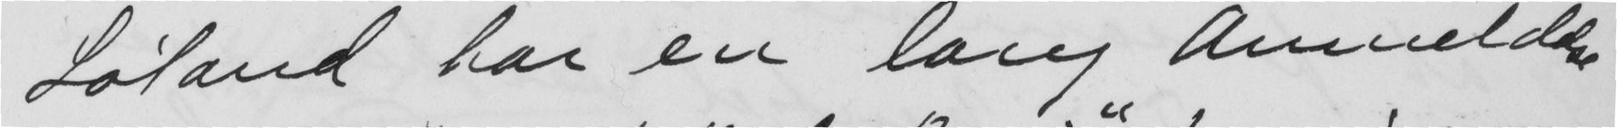Løland har en lang Anmeldelse
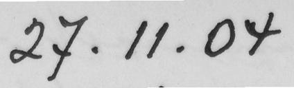27.11.04
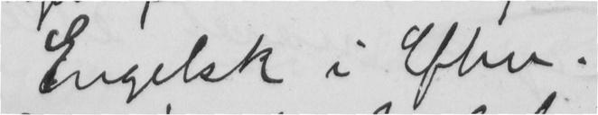Engelsk i Eften.
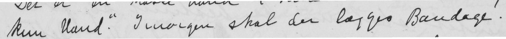kun Vand". Imorgen skal der lægges Bandage.
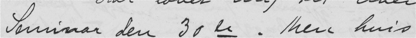Semiar den 30te. Men hvis
